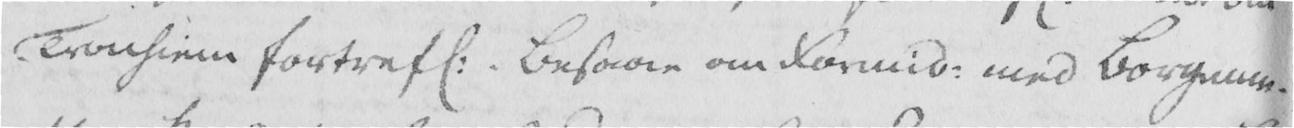Tronhiem fortrefl. Besaae om formid. med Borgerme-
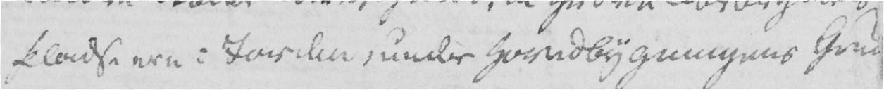Pladse ere i Jorden, under Hovedbygningens Grund
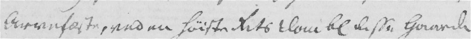Arvefæste, ved en høiste Rets Dom bl disse Gaarde
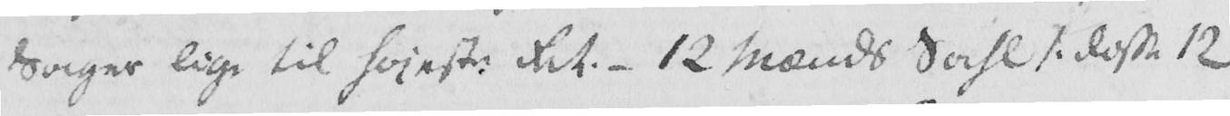Sager lige til høieste Ret. - 12 Mænds Sahl /: disse 12
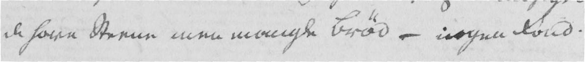de have Steene men mangle Brød - ingen Fond.
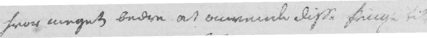hvor meget bedre at anvende disse Penge til
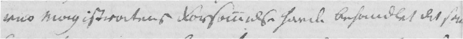ved Magistratens Forsømelse havde behandlet det som
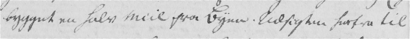bygget en halv Miil fra Byen. Udsigten herfra til
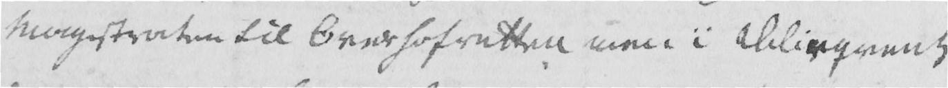Magistraten til Overhofretten men i Deliqvent
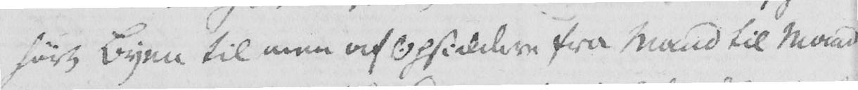hørt Byen til men af Opsidderne fra Mand til Mand
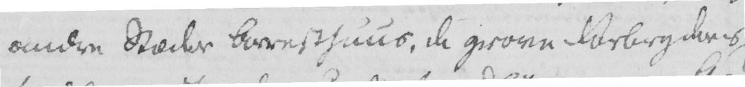andre Stæder Arresthuus, de grove Forbryderes
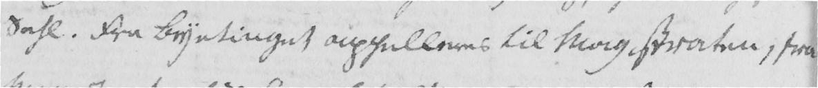Sahl. Fra Byetinget appelleres til Magistraten, fra
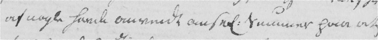af nogle havde anvendt ansel. Summer paa at
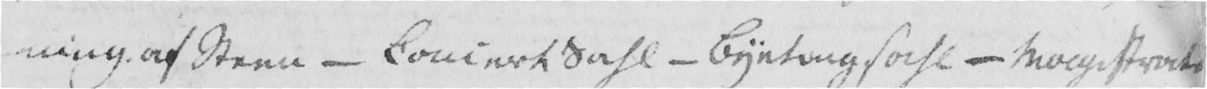ning af Steen - Consert Sahl - Byetingsahl - Magistrats
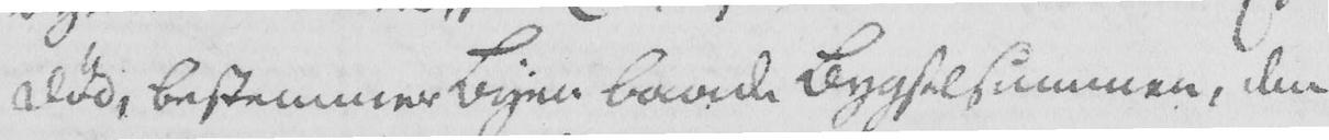Død, bestemmer Byen baade Bygselsummen, den
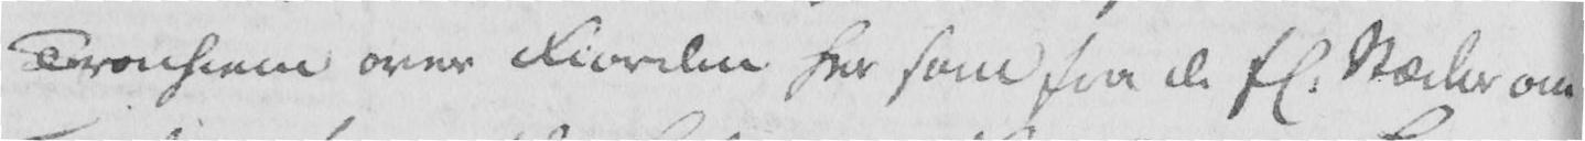Tronhiem over Fiorden her som fra de fl. Stæder om
